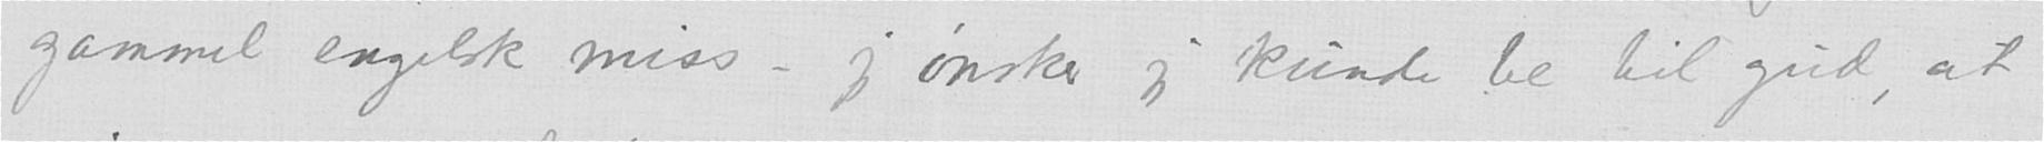gammel engelsk miss - jeg ønsker jeg kunde be til gud, at
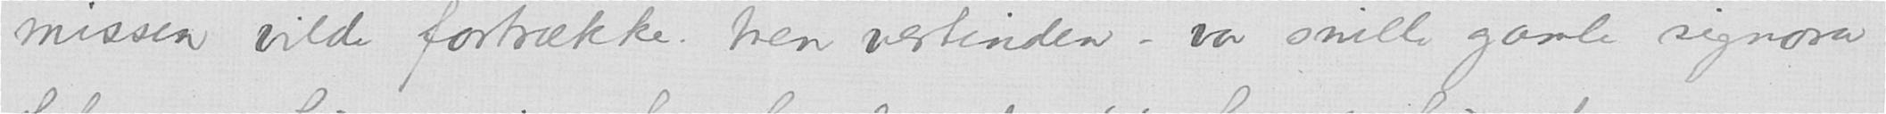missen vilde fortrække. Men vertinden, vor snille gamle signora
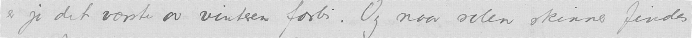er jo det værste av vinteren forbi. Og naar solen skinner findes
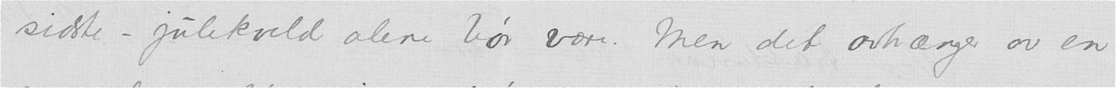sidste - julekveld alene bør være. Men det avhænger av en
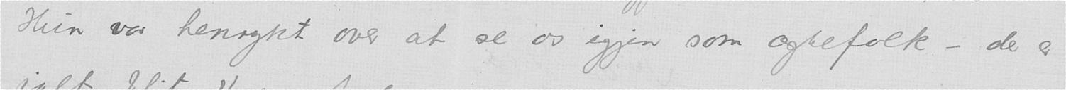Hun var henrykt over at se os igjen som ægtefolk - der er
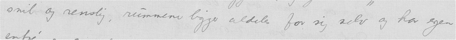snil og renslig, rummene ligger aldeles for sig selv og har egen
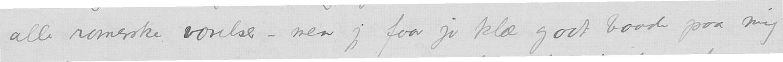alle romerske værelser - men jeg faar jo klæ godt baade paa mig
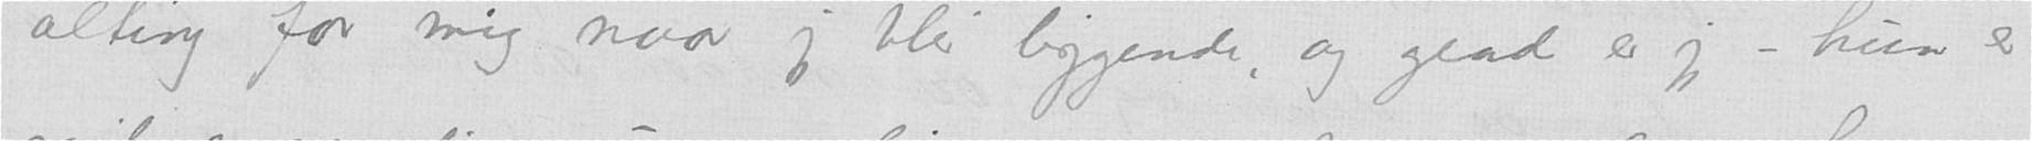alting for mig naar jeg blir liggende, og glad er jeg - hun er
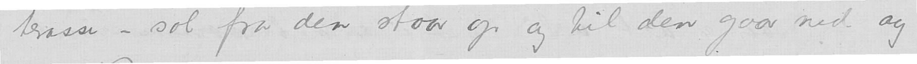terasse - sol fra den staar op og til den gaar ned og
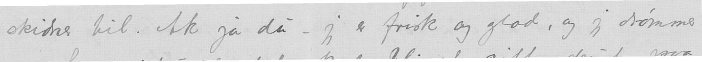skidner til. Ak ja du - jeg er frisk og glad, og jeg drømmer
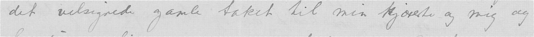det velsignede gamle taket til min kjæreste og mig og
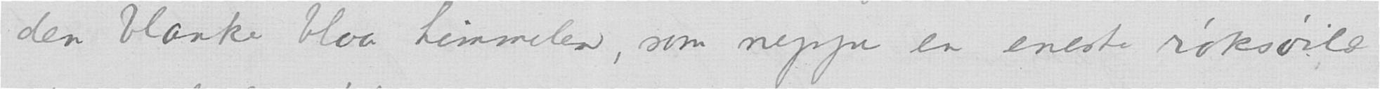den blanke blaa himmelen, som neppe en eneste røksøile
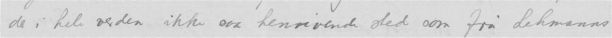der i hele verden ikke saa henrivende sted som fru Lehmanns
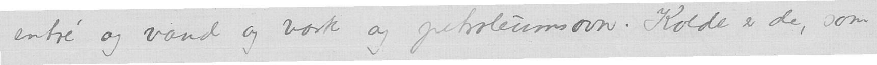entré og vand og vask og petroleumsovn. Kolde er de, som
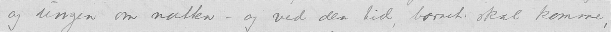og ungen om natten - og ved den tid, barnet skal komme,
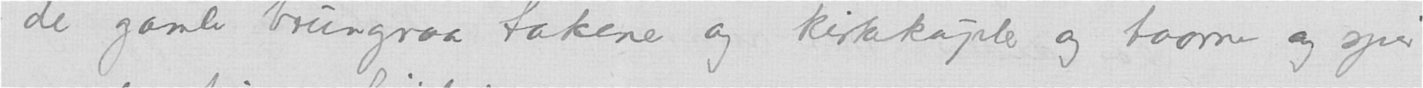de gamle brungraa takene og kirkekupler og taarn og spir
